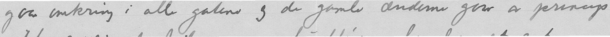gaar omkring i alle gatene og de gamle ænderne gaar av princip
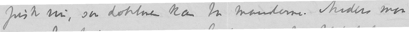frisk nu, saa doktoren kan ta mandlene. Anders maa
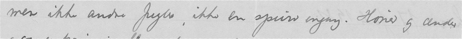men ikke andre fugler, ikke en spurv engang. Høner og ænder
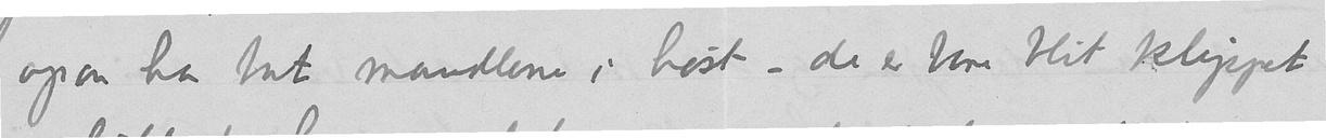ogsaa ha tat mandlene i høst – de er bare blit klippet
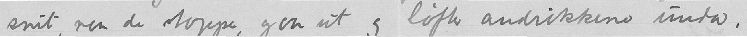snit, naar de stopper, gaar ut og løfter andrikkene unda,
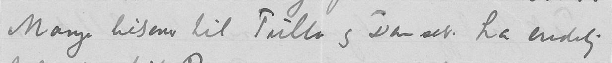Mange hilsener til Tulla og Dem selv. La endelig
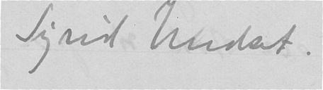Sigrid Undset.
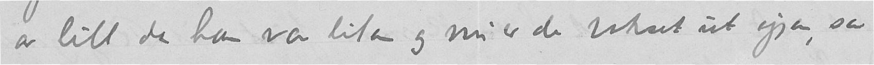av litt da han var liten og nu er de vokset ut igjen, sa
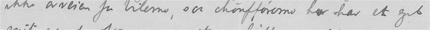ikke avveien for bilerne, saa choufførerne her har et eget
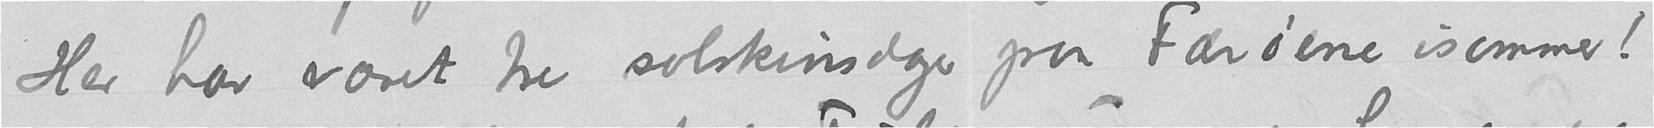Her har været tre solskinsdager paa Færøene isommer!
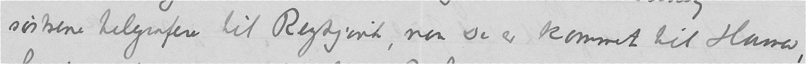søstrene telegrafere til Reykjavik, naar De er kommet til Hamar,
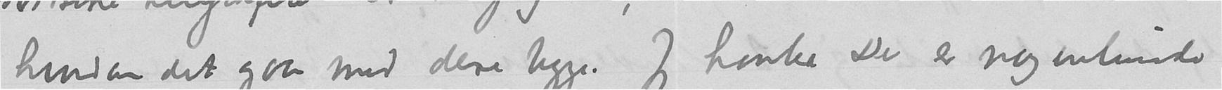hvordan det gaar med dere begge. Jeg tenker De er nogenlunde
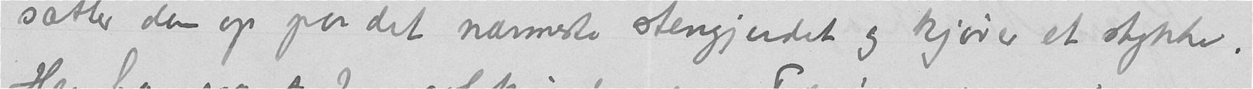sætter dem op paa det narmeste stengjerdet og kjører et stykke.
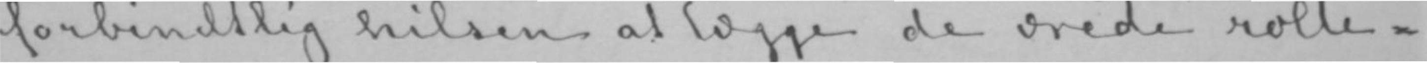forbindtlig hilsen at lægge de ærede rolle-
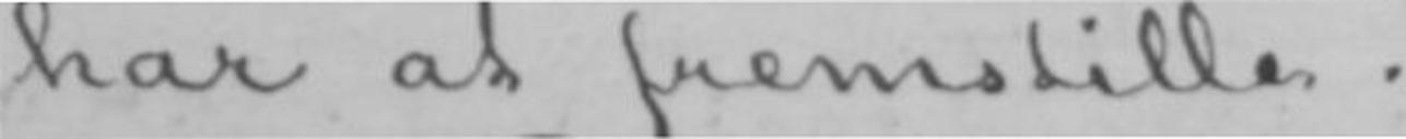har at fremstille.
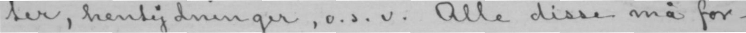ter, hentydninger, o. s. v. Alle disse må for-
